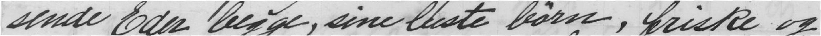sende Eder, sine bedste børn, friske og
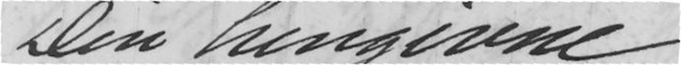Din hengivne
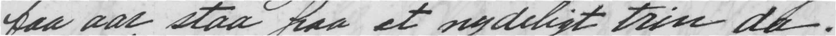faa aar staa paa et nydeligt trin da.
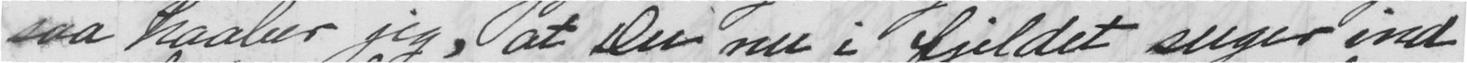saa haaber jeg, at Du nu i fjeldet suger ind
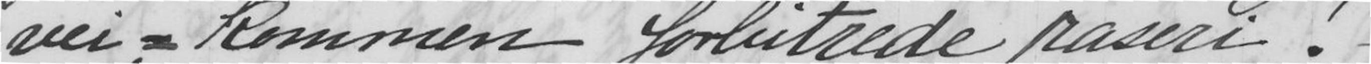vei=kommen forbitrede raseri!
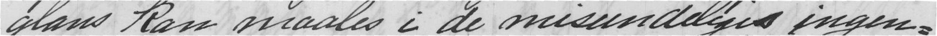glans kan maales i de misundeliges ingen-
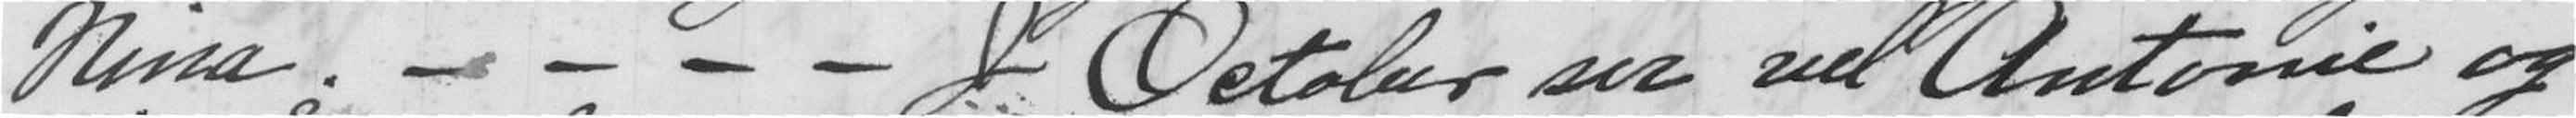Nina. - - - I October ser vel Antonie og
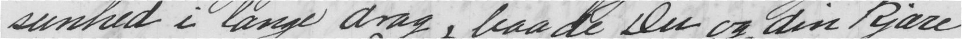sunhed i lange drag, baade Du og din kjære
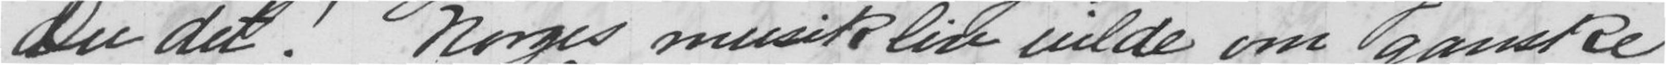Du det! Norges musikliv vilde om ganske
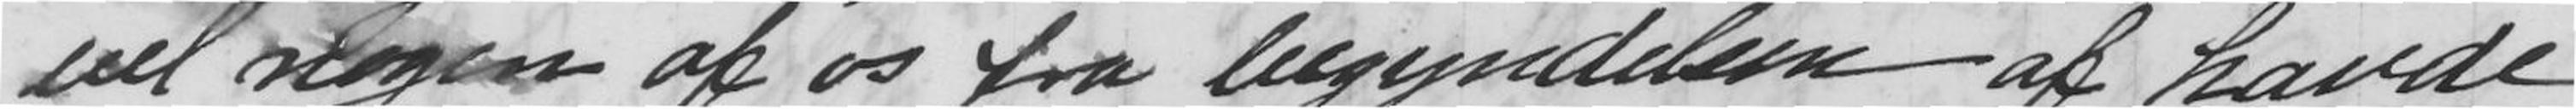vel nogen af os fra begyndelsen af havde
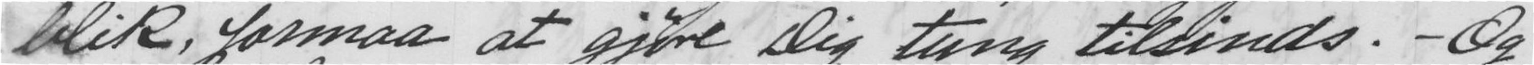blik, formaa at gjøre Dig tung tilsinds. - Og
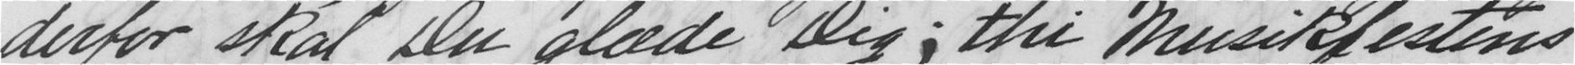derfor skal Du glæde Dig; thi Musikfestens
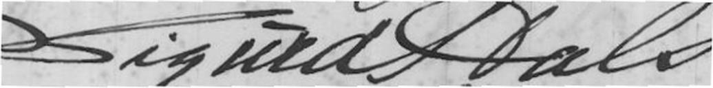Sigurd Hals.
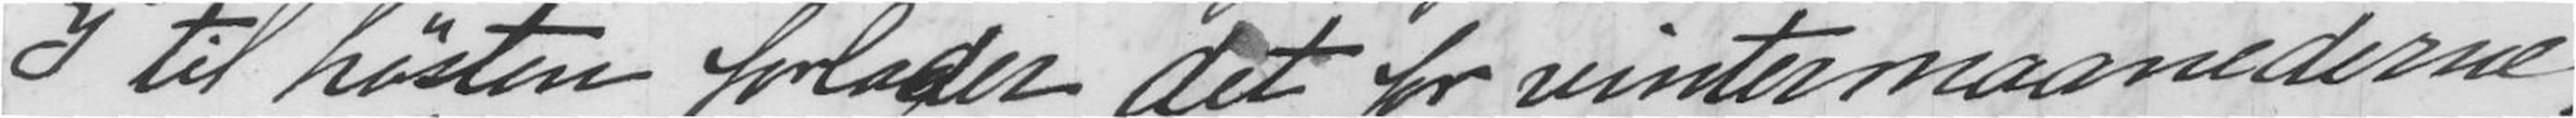I til høsten forlader det for vintermaanederne
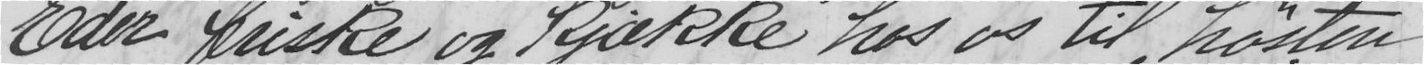Eder friske og kjække hos os til høsten.
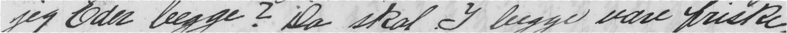jeg Eder begge? Da skal I begge være friske.
